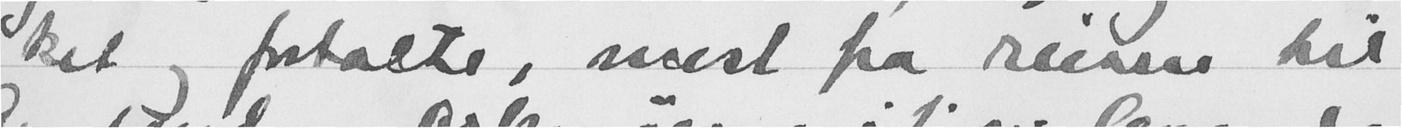ket og fortalte, mest fra reisen til
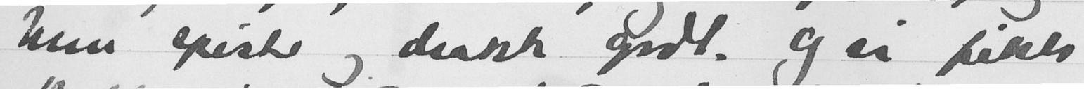hun spiste og drakk godt og vi fikk
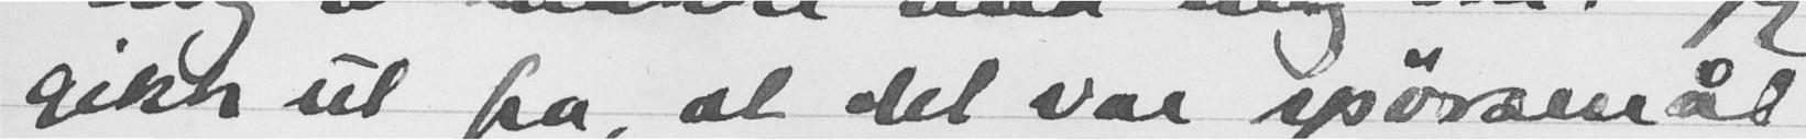gikk ut fra, at det var spørsmål
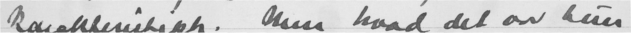karakteristikk. Men hvad det var hun
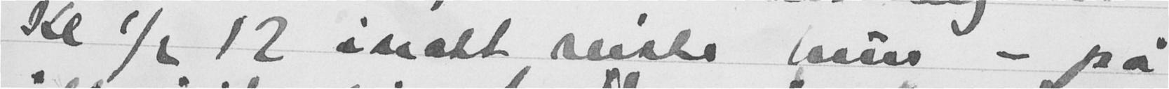Kl 1/2 12 inatt reiste hun - på
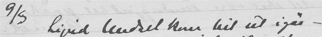9/5 Sigrid Undset kom hit ut igår -
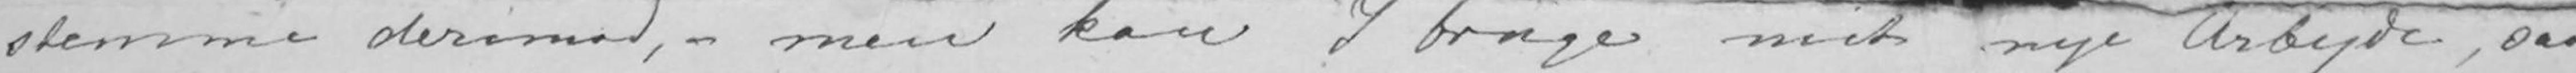stemme derimod,- men kan I bruge mit nye Arbejde, saa
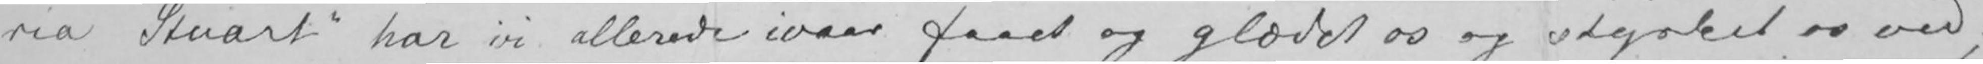ria Stuart» har vi allerede ivaar faaet og glædet os og styrket os ved;
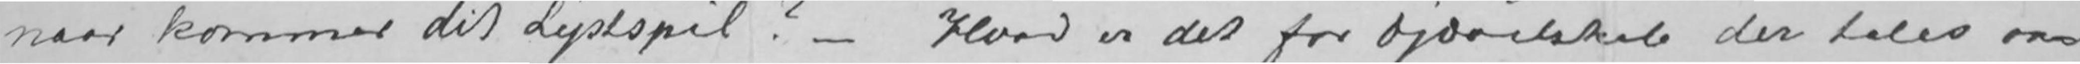naar kommer dit Lystspil?- Hvad er det for Djævelskab der tales om-
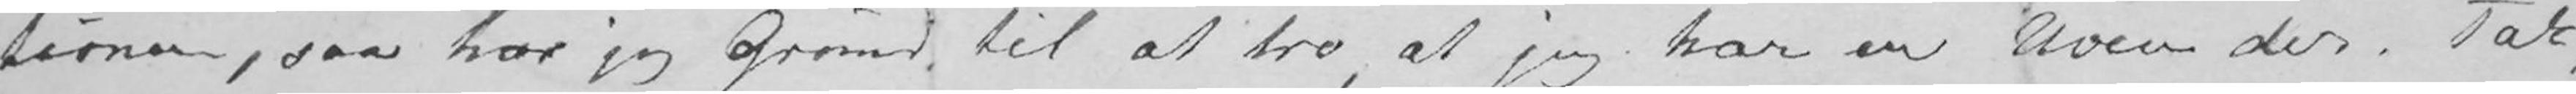tionen, saa har jeg Grundgrund til at tro, at jeg har en Uven der. Tak,
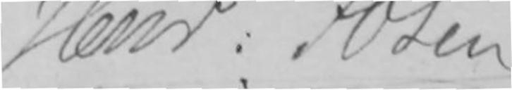Henr: Ibsen
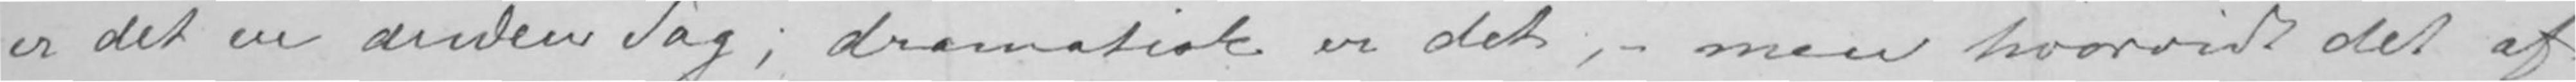er det en anden Sag; dramatisk er det,- men hvorvidt det af
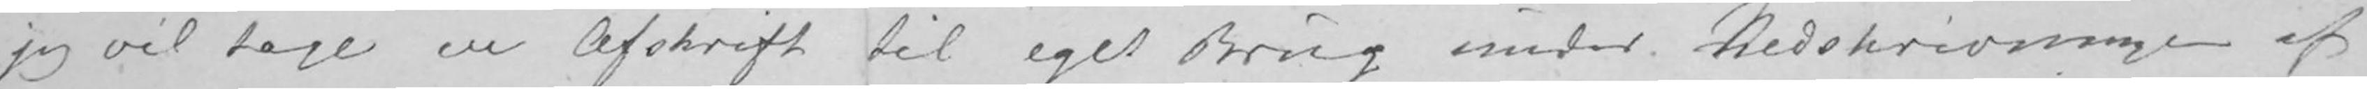jeg vil tage en Afskrift til eget Brug under Nedskrivningennedskrivningen af
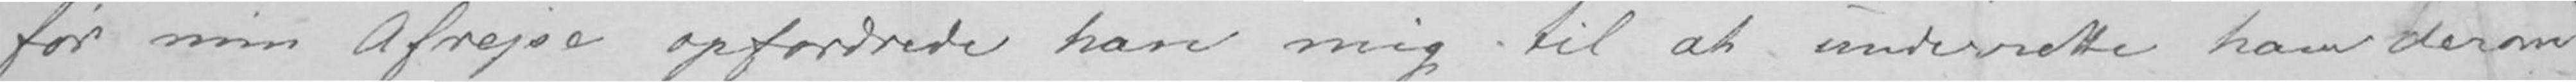før min Afrejse opfordrede han mig til at underrette ham derom
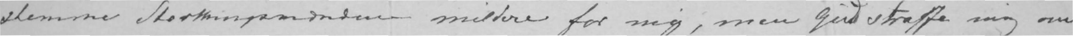stemme Storthingsmændene mildere for mig, men Gud straffe mig om
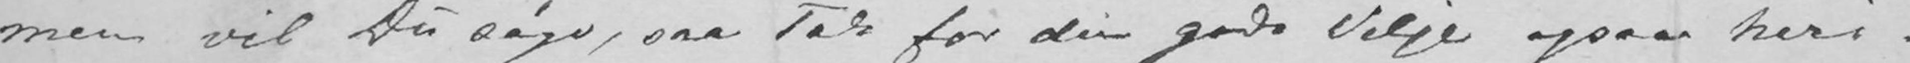men vil Du søge, saa Tak for din gode Vilje ogsaa heri.
